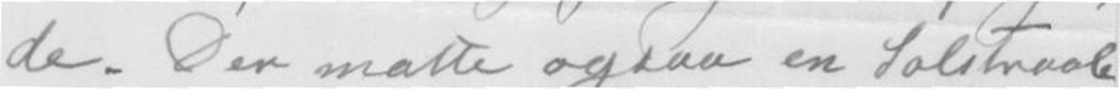de. Der matte ogsaa en Solstraale
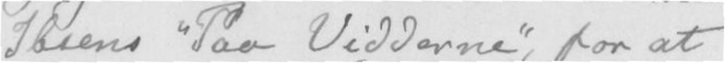Ibsens "Paa Vidderne", for at
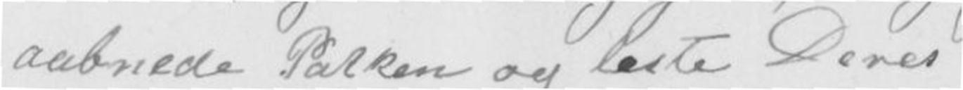aabnede Pakken og leste Deres
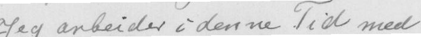Jeg arbeider i denne Tid med
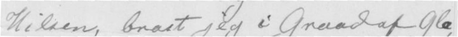Hilsen, brast jeg i Graad af glæ-
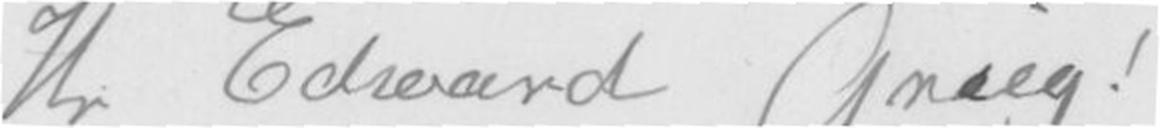Hr Edvard Grieg!
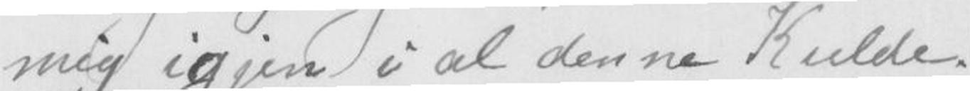mig igjen i al denne Kulde.
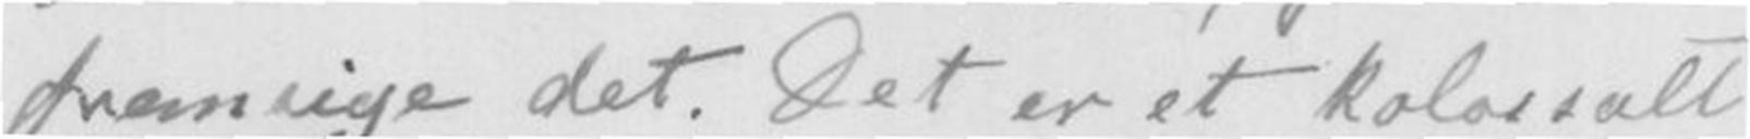framsige det. Det er et kolossalt
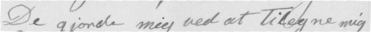De gjorde mig ved at tilegne mig
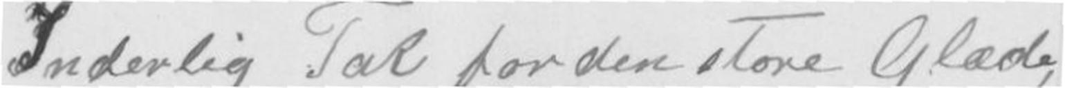Inderlig Tak for den store Glæde,
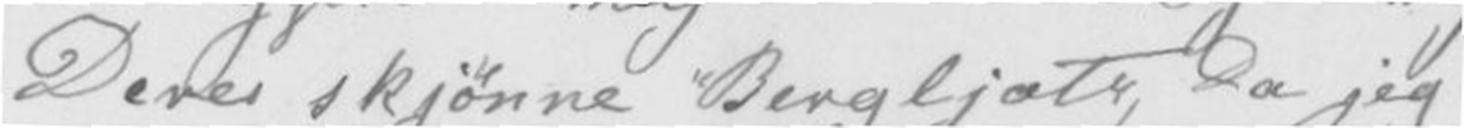Deres skjønne Bergljot, Da jeg
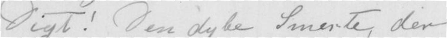Digt! Den dybe smerte, der
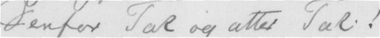Derfor Tak og atter Tak!
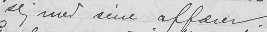slig med sine affærer.
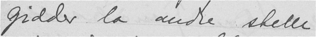gidder la andre stelle
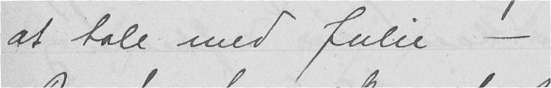at tale med Julie -
